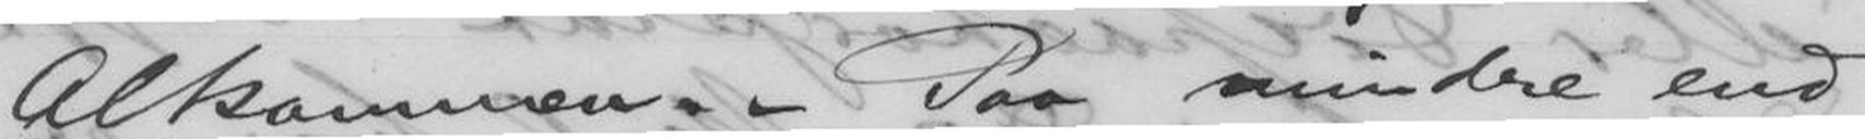Altsammen. - Paa mindre end
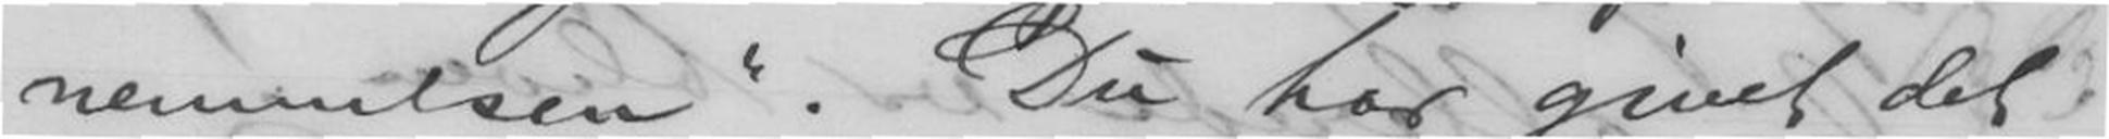næmmelsen". Du har givet det
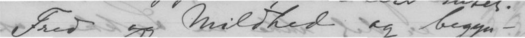Fred og Mildhed og begyn-
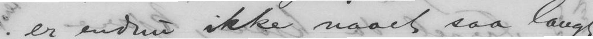er endnu ikke naaet saa langt
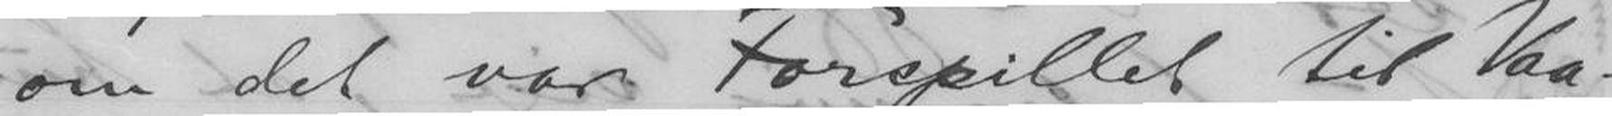om det var Forspillet til Vaa-
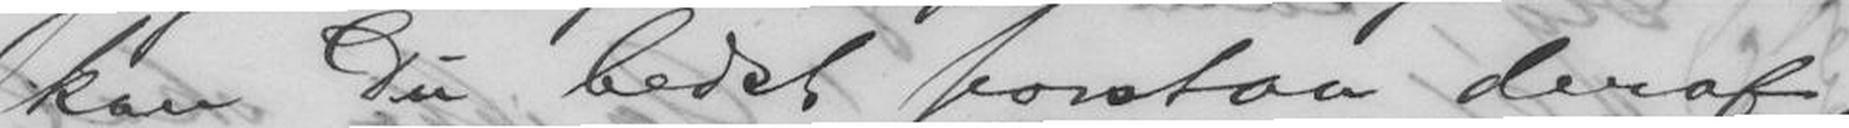kan Du bedst forstaa deraf,
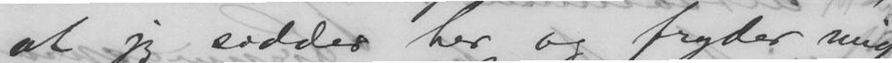at jeg sidder her og fryder mig
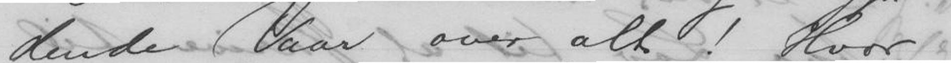dende Vaar over alt! Hvor
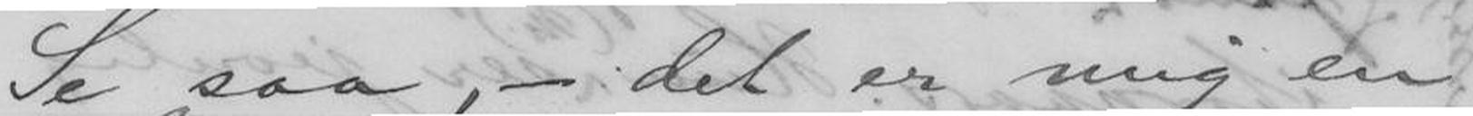Se saa, - det er mig en
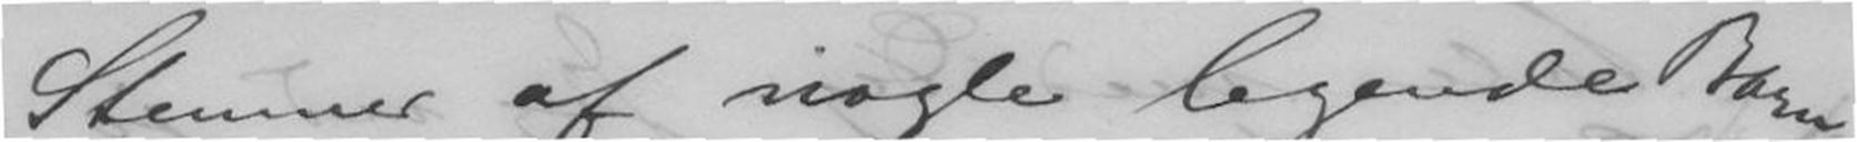Stemmer af nogle legende Børn
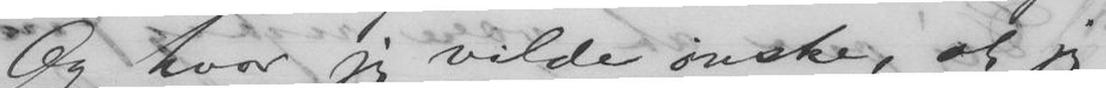Og hvor jeg vilde ønske, at jeg
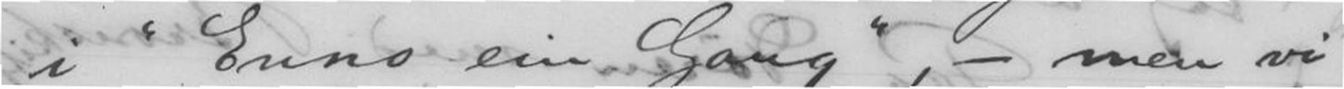i "Enno ein Gong", - men vi
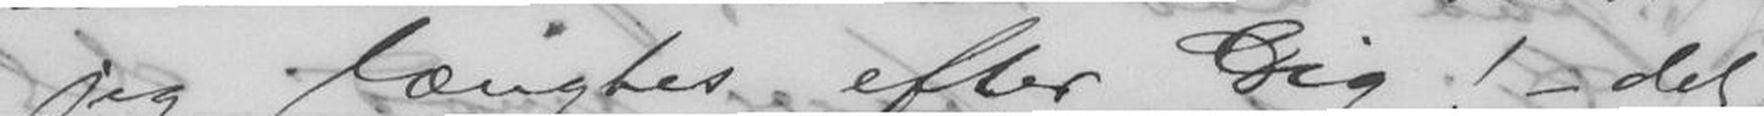jeg længtes efter Dig! - det
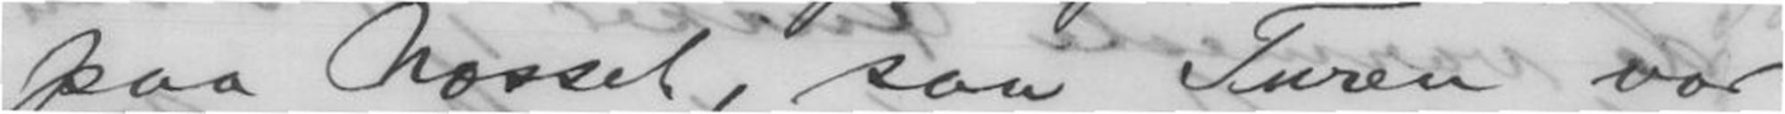paa Næsset, saa Turen var
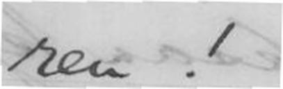ren!
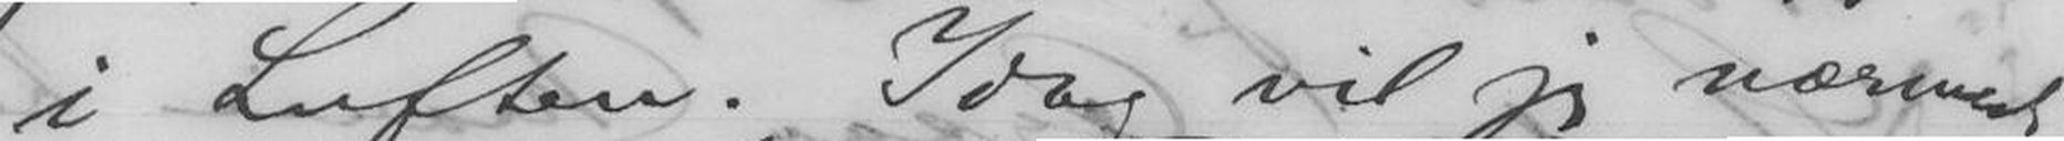i Luften. Idag vil jeg nærmest
